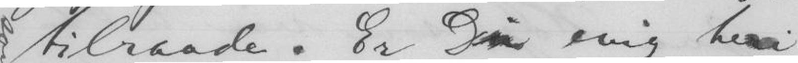tilraade. Er Du enig heri
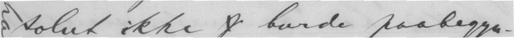solut ikke burde paabegyn-
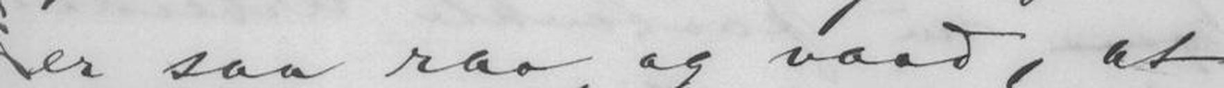er saa raa og vaad, at
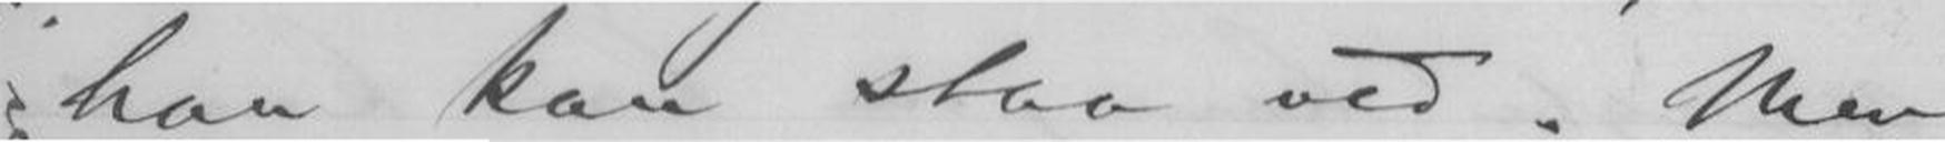han kan staa ved. Men
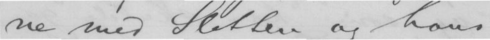ne med Sletten og hans
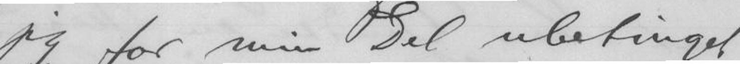jeg for min Del ubetinget
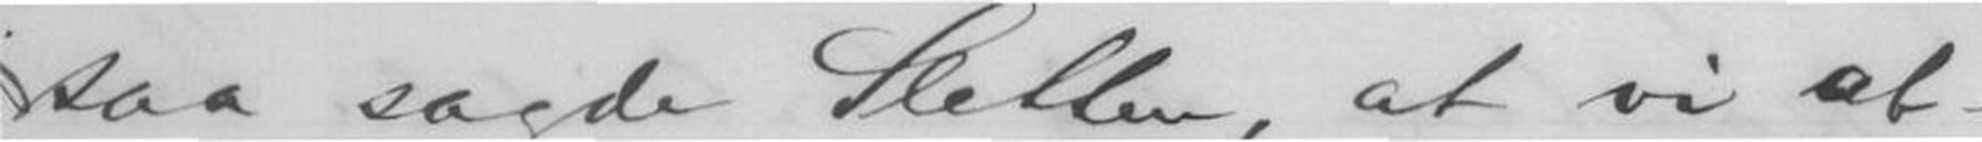saa sagde Sletten, at vi ab-
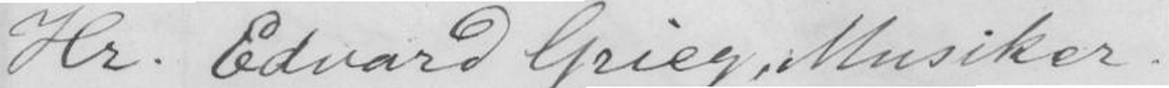Hr. Edvard Grieg, Musiker.
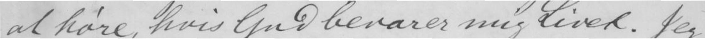at høre, hvis Gud bevare mig Livet. Jeg
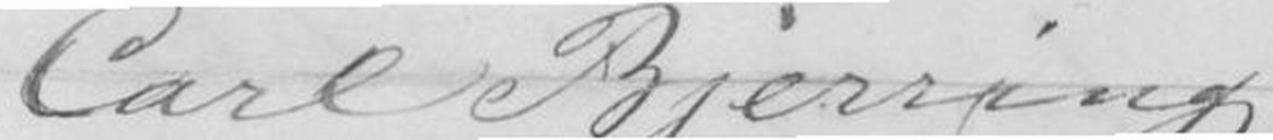Carl Bjerring
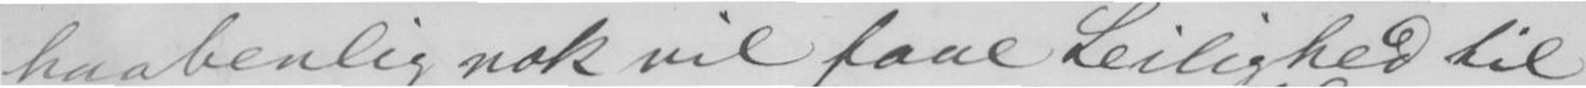haabenlig nok vil faae Leilighed til
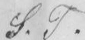S.T.
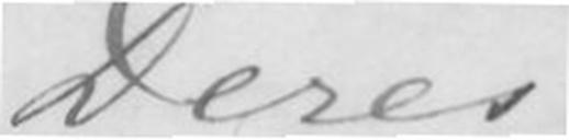Deres
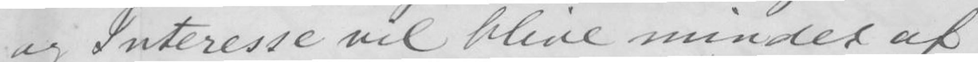og Interesse vil blive mindet af
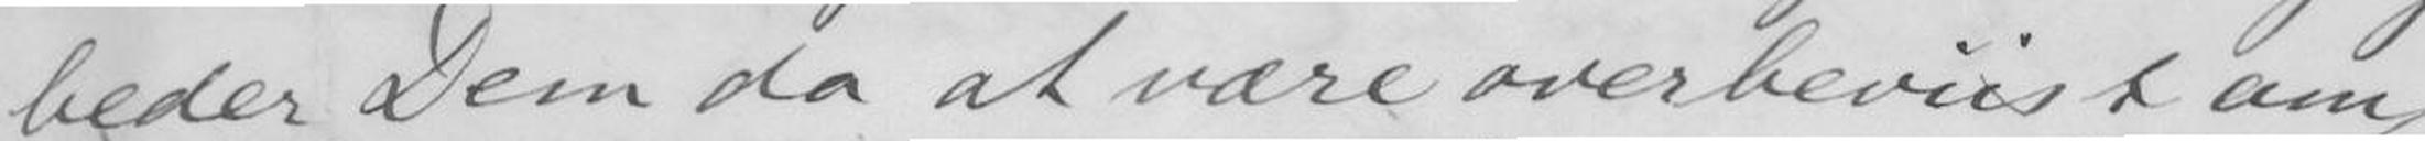beder Dem da at være overbeviist om,
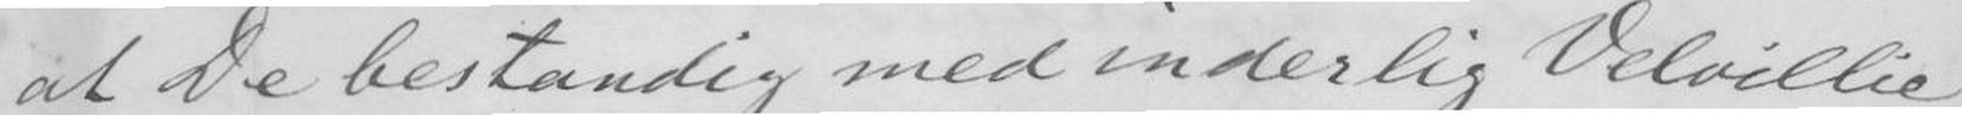at De bestandig med inderlig Velvillie
